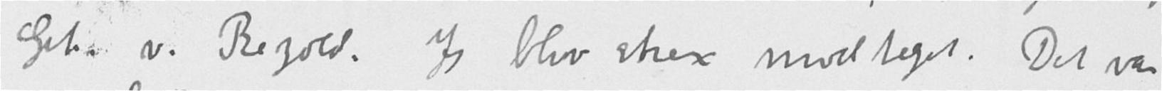Geh. v. Bezold. Jeg blev strax modtaget. Det var
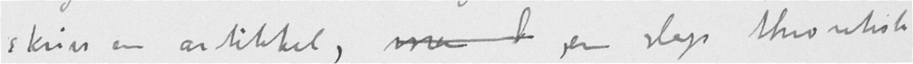skrive en artikkel, men er en slags throntisk
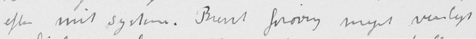efter mit system. Brevet forøvrig meget venligt
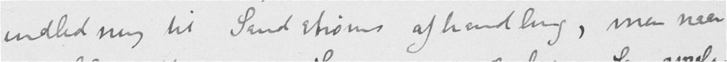indledning til Sandstrøms afhandling, men naar
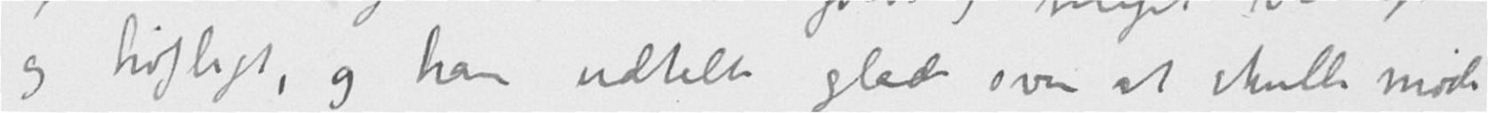og høfligt, og han udtalte glæde over at skulle møde
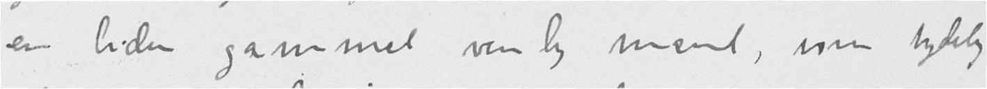en liden gammel venlig mand, som tydelig
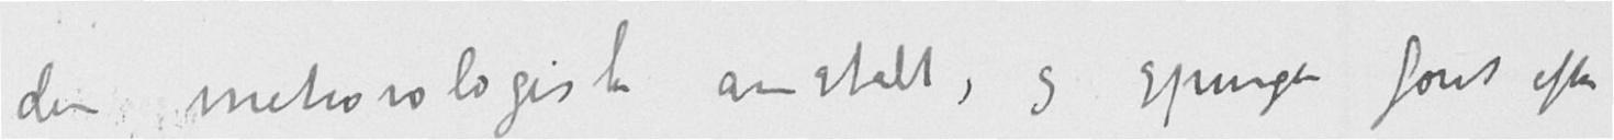den meteorologiske anstalt, og spurgte først efter
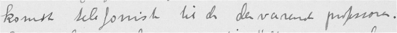komst telefonisk til de derværende professorer.
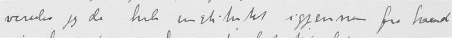veredes jeg da hele institutet igjennem fra haand
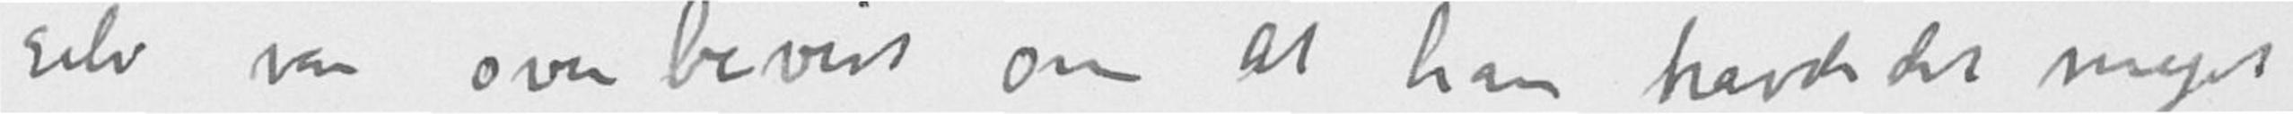selv var overbevist om at han havde det meget
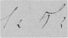S: T:
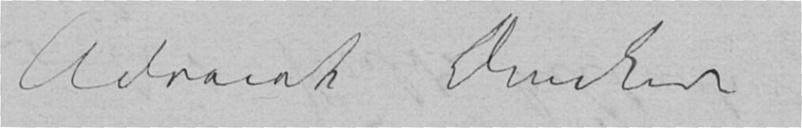Advocat Dunker.
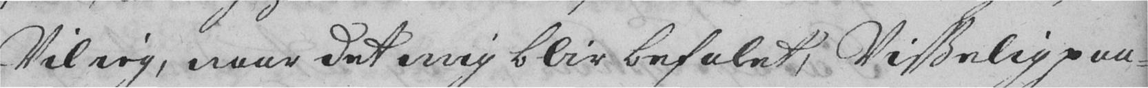Vil ieg, naar det mig blir befalet, Visselig paa-
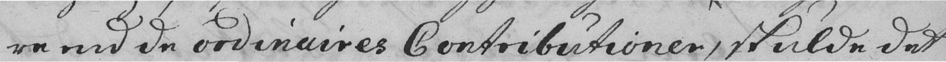re end de ordinaires Contributioner, skulde det
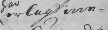erlagt me-
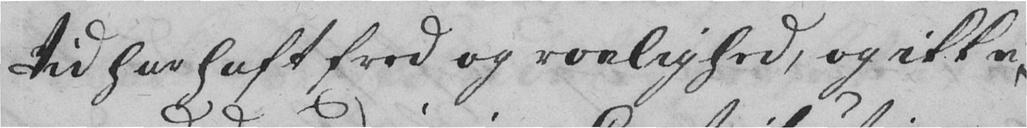tid har haft fred og roelighed, og ikke
